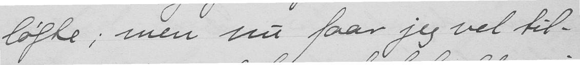løfte; men nu faar jeg vel til-
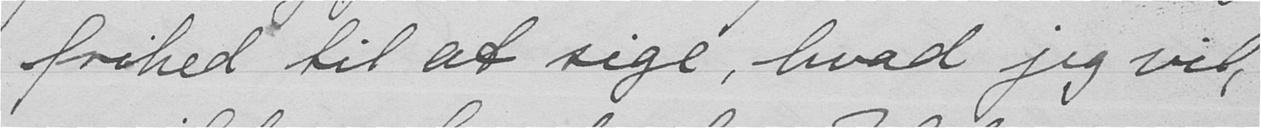frihed til at sige, hvad jeg vil,
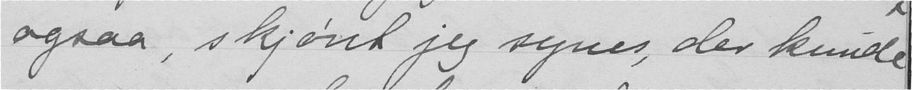ogsaa, skjønt jeg synes, der kunde
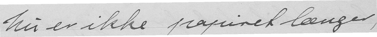Nu er ikke papiret længer,
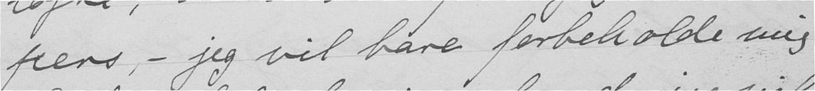pers, - jeg vil bare forbeholde mig
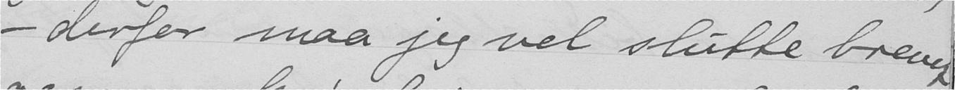- derfor maa jeg vel slutte brevet
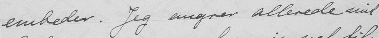embeder. Jeg angrer allerede mit
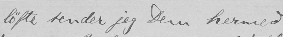løfte sender jeg Dem hermed
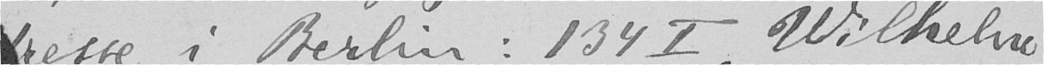resse i Berlin: 134 I, Wilhelm-
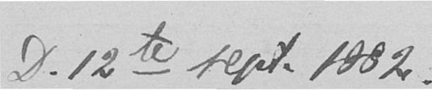D. 12te sept. 1882.
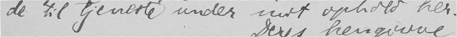de til tjeneste under mit ophold her.
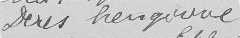Deres hengivne
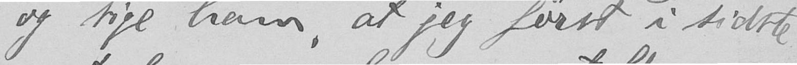og sige ham, at jeg først i sidste
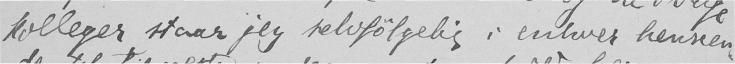kolleger staar jeg selvfølgelig i enhver henseen-
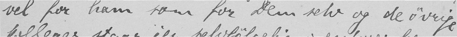vel for ham som for Dem selv og de øvrige
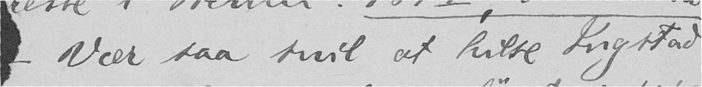- Vær saa snil at hilse Ingstad
